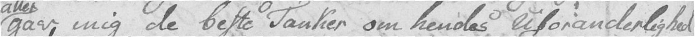atter gav mig de beste Tanker om hendes uforanderlighed
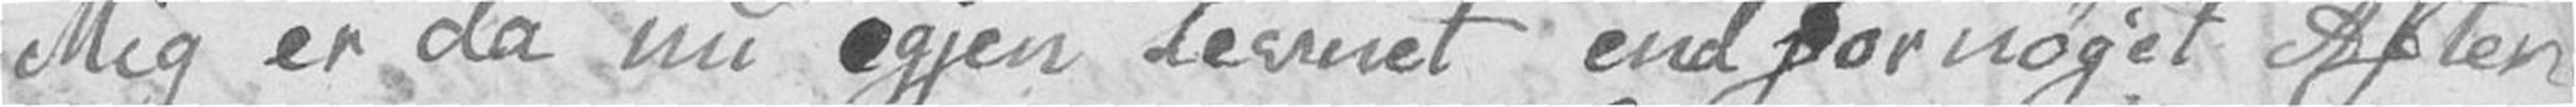Mig er da nu igjen levnet end fornøjet Aften.
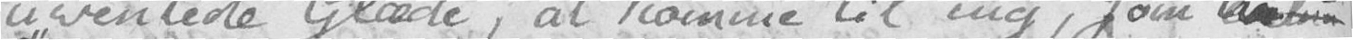uventede Glæde, at komme til mig, som
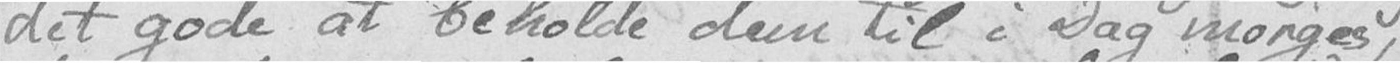det gode at beholde dem til i Dag morges,
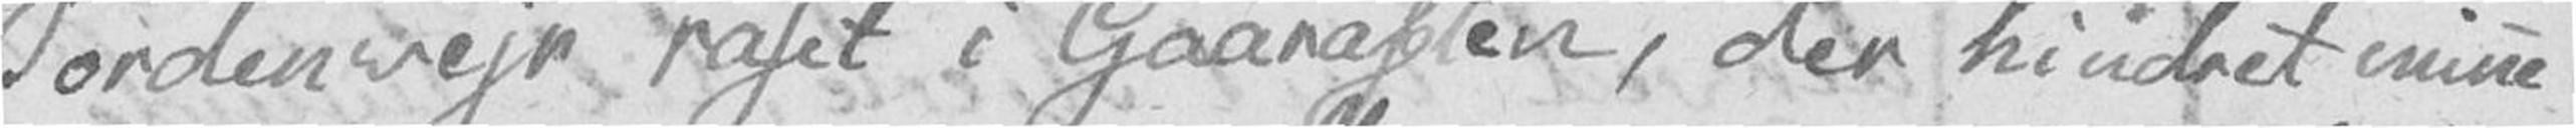Tordenvejr raset i Gaaraften, der hindret mine
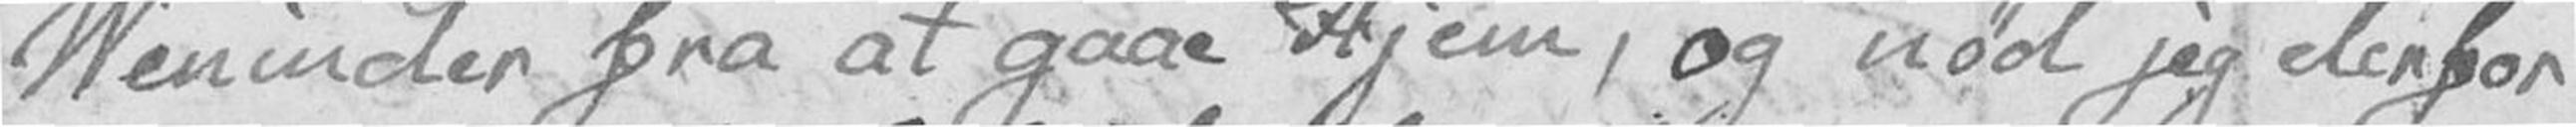Veninder fra at gaae Hjem, og nød jeg derfor
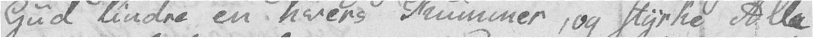Gud lindre en hvers Kummer, og styrke Alle
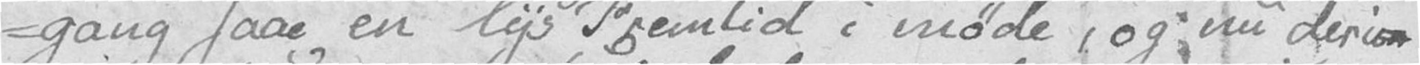-gang saae en lys Fremtid i møde, og nu deri
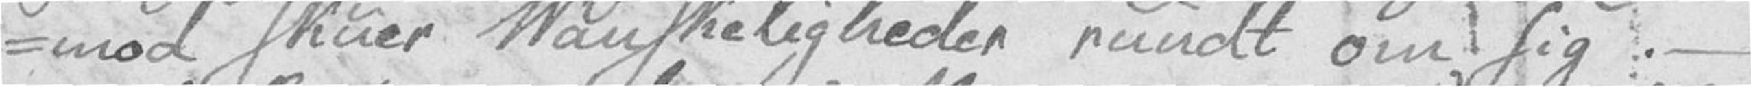-mod skuer Vanskeligheder rundt om sig. –
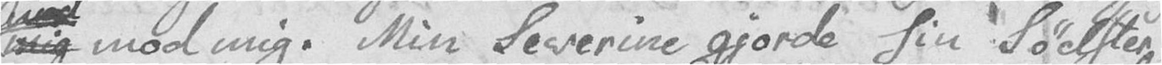mod mig mod mig. Min Severine gjorde sin Sødster
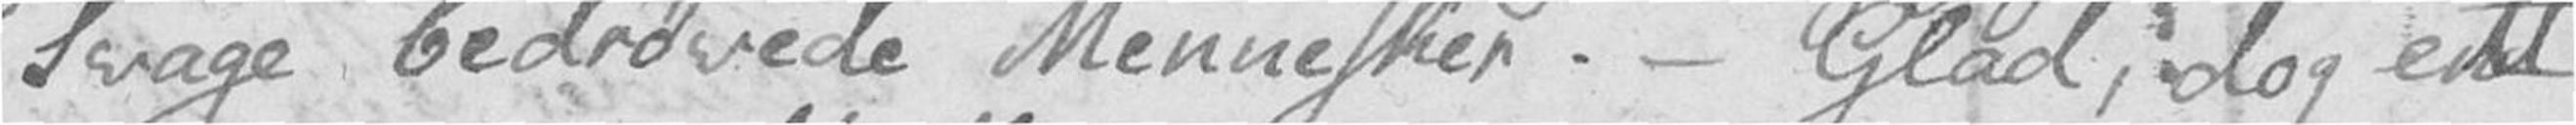Svage bedrøvede Mennesker. – Glad, dog ett
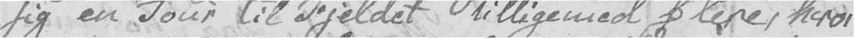sig en Tour til Fjeldet tilligemed flere, hvor
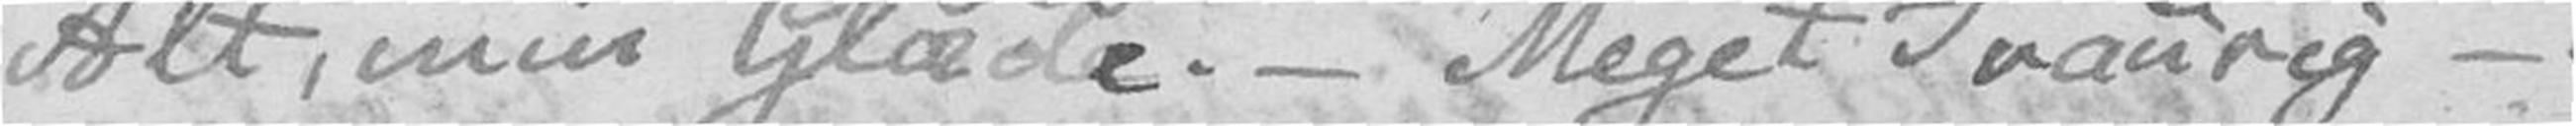Alt, min Glæde. – Meget Traurig –
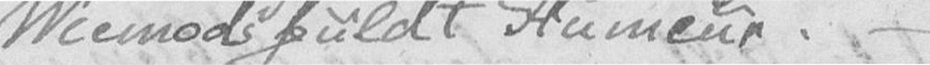Veemodsfuldt Humeur. –
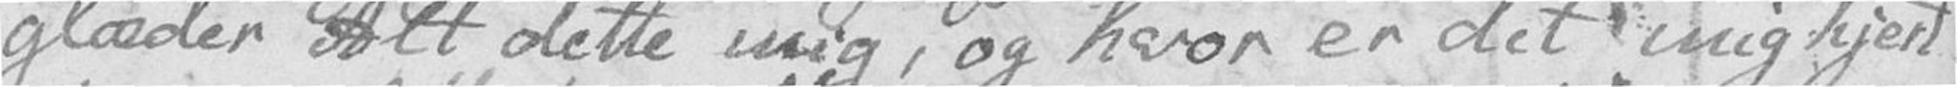glæder Alt dette mig, og hvor er det mig kjert
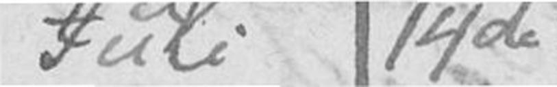Juli 14de
